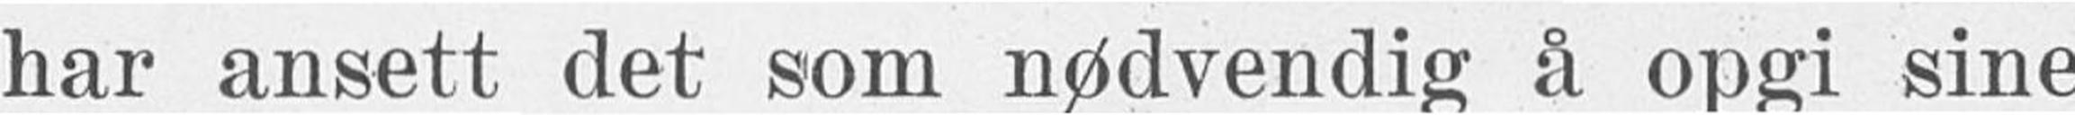har ansett det som nødvendig å opgi sine
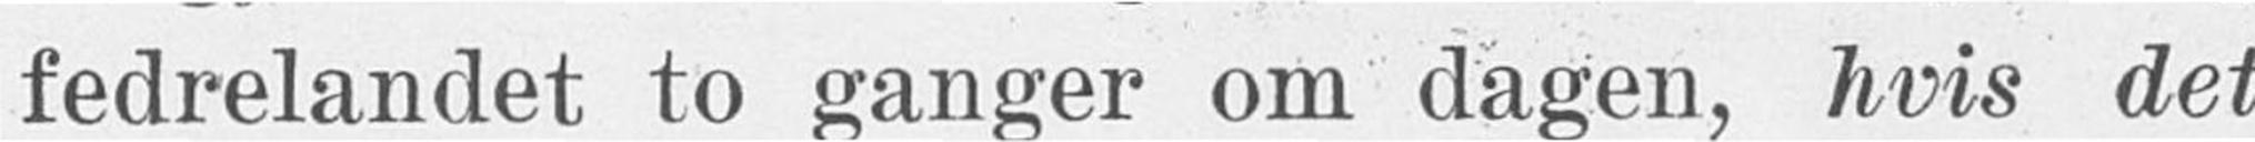fedrelandet to ganger om dagen, hvis det
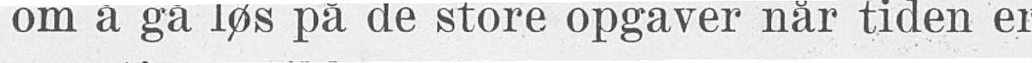om å gå løs på de store opgaver når tiden er
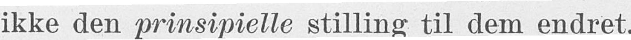ikke den prinsipielle stilling til dem endret.
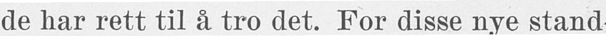de har rett til å tro det. For disse nye stand-
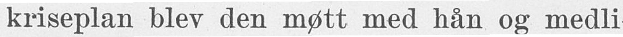kriseplan blev den møtt med hån og medli-
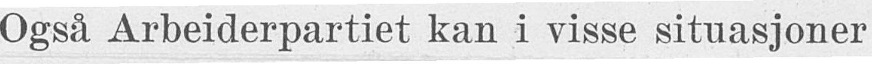Også Arbeiderpartiet kan i visse situasjoner
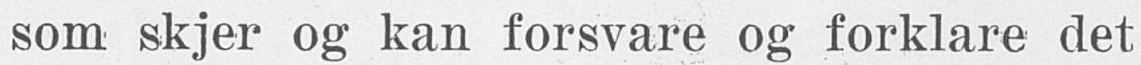som skjer og kan forsvare og forklare det
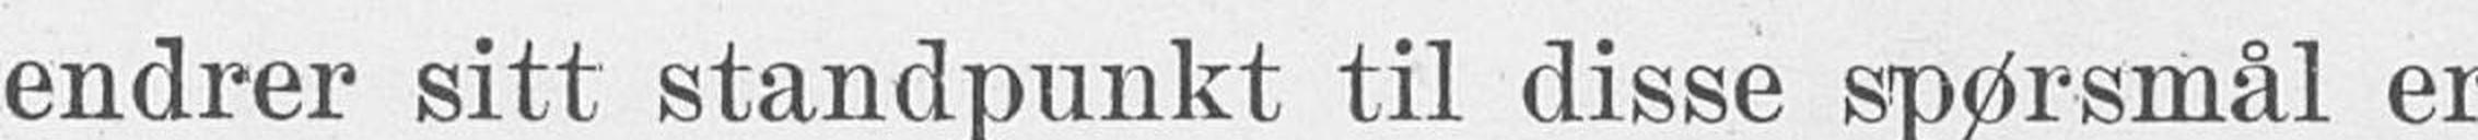endrer sitt standpunkt til disse spørsmål er
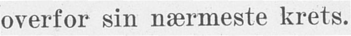overfor sin nærmeste krets.
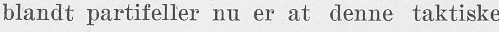blandt partifeller nu er at denne taktiske
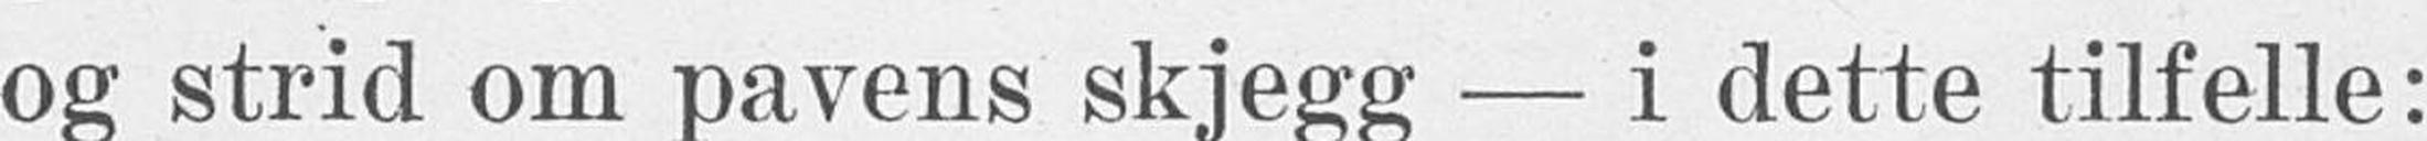og strid om pavens skjegg - i dette tilfelle:
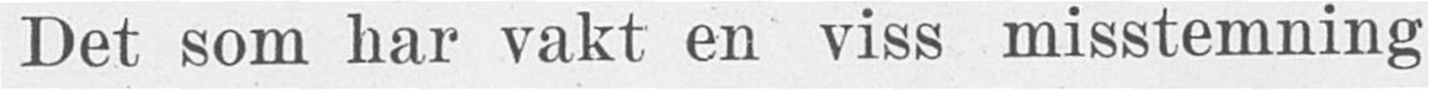Det som har vakt en viss misstemning
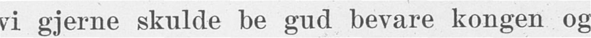vi gjerne skulde be gud bevare kongen og
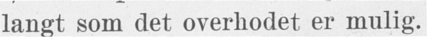langt som det overhodet er mulig.
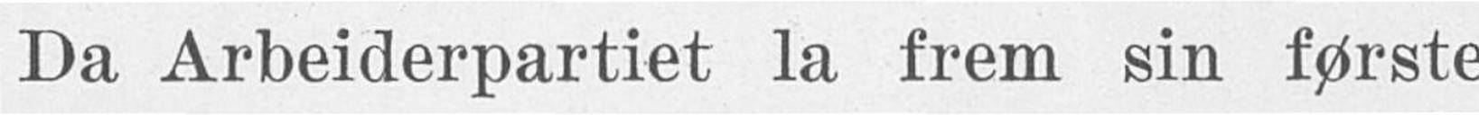Da Arbeiderpartiet la frem sin første
